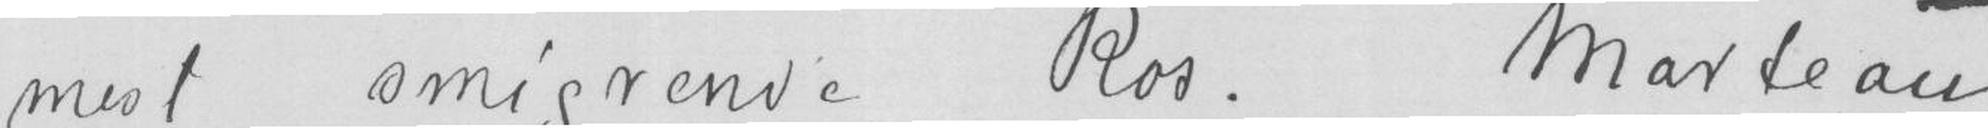mest smigrende Ros. Marteau
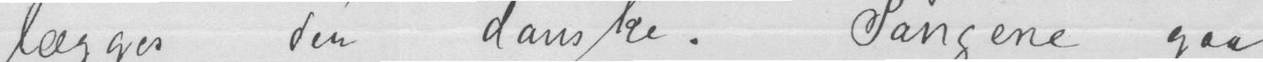lægges den danske , Sangene gaa
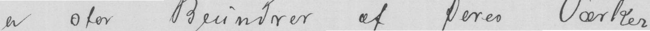er stor Beundrer af Deres Værker
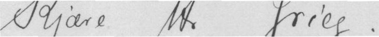Kjære Hr. Grieg!
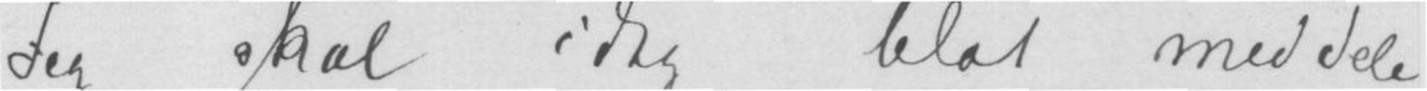Jeg skal idag blot meddele
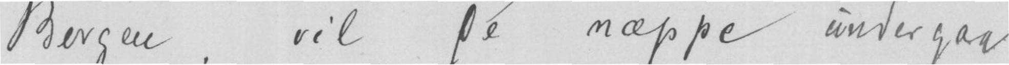Bergen, vil De næppe undergaa
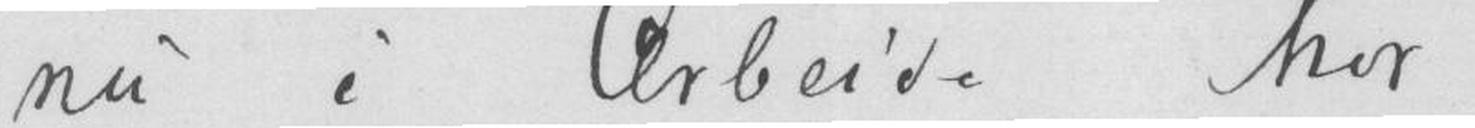nu i Arbeide her
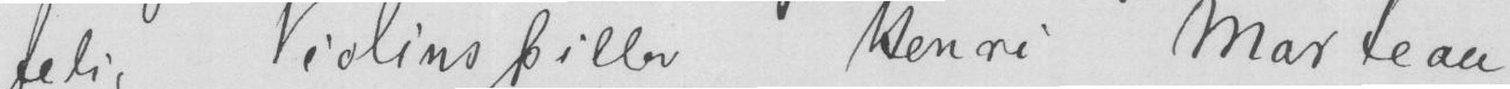felig Violinspiller Henri Marteau
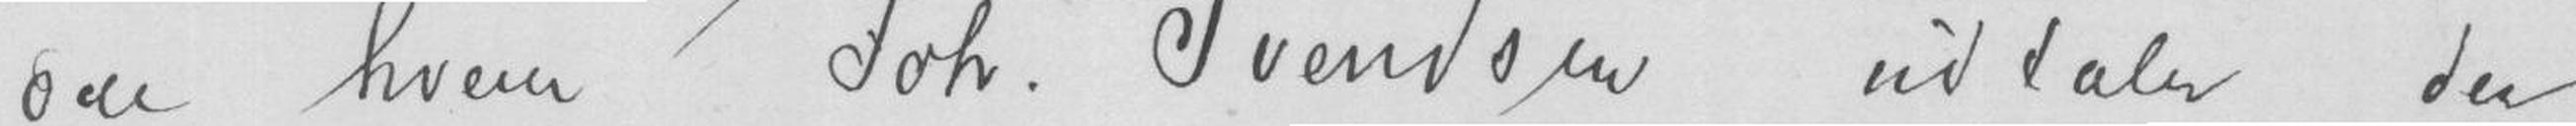ou hvem Joh. Svendsen udtaler der
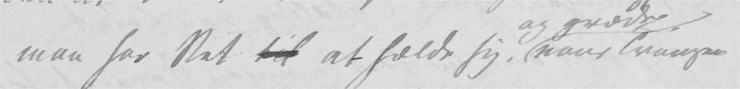man har Ret til at hælde sig, naar Trangen
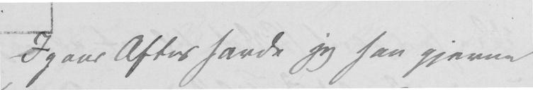Igaar Aftes havde jeg saa gjerne
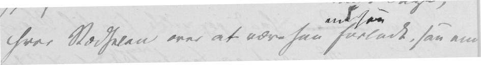hvor Rædselen over at være saa forladt, saa ene
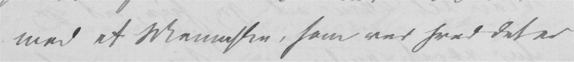med et Menneske, som ved hvad det er
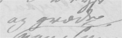og græde
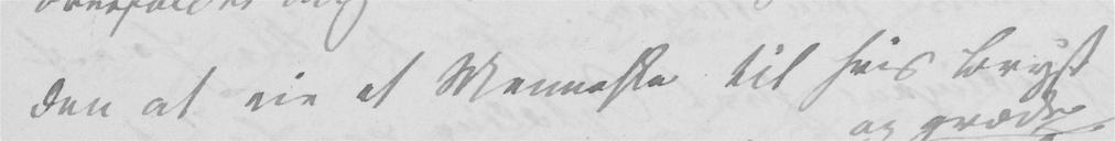den at eie et Menneske til hvis Bryst
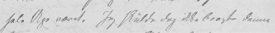hele Uge været. Jeg skulde dog ikke bragt denne
